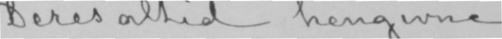Deres altid hengivne
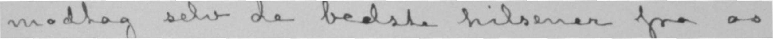modtag selv de bedste hilsener fra os
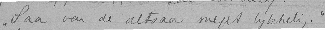"Saa var de altsaa meget lykkelig."
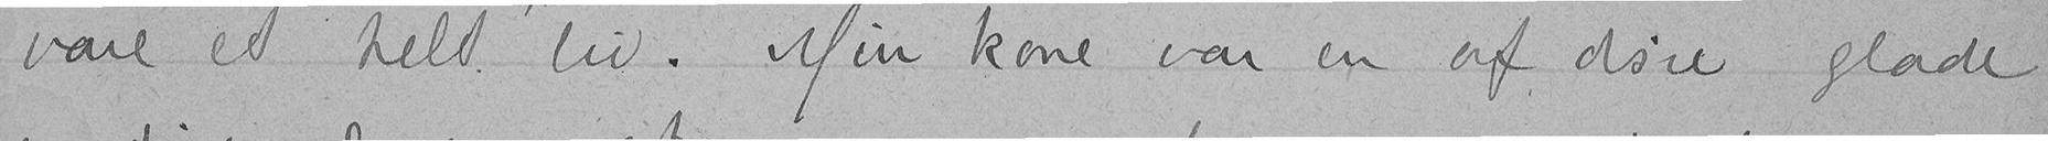vare et helt liv. Min kone var en af disse glade
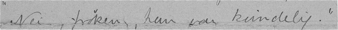"Nei, frøken, hun var kvindelig."
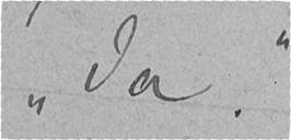"Ja."
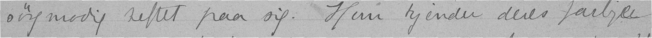sørgmodig heftet paa sig. Hun kjender deres farlige
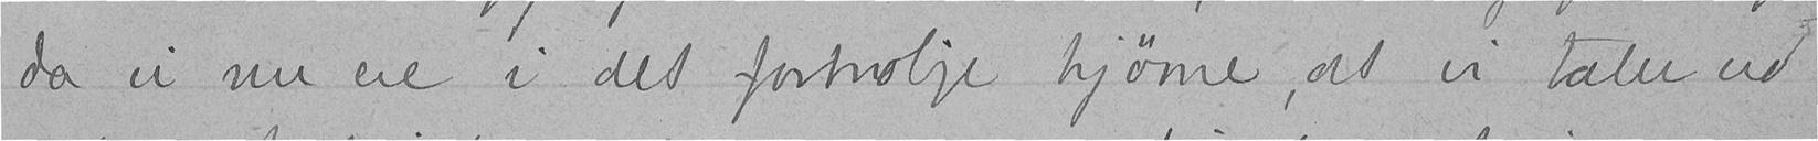da vi nu ere i det fortrolige hjørne, at vi taler ud
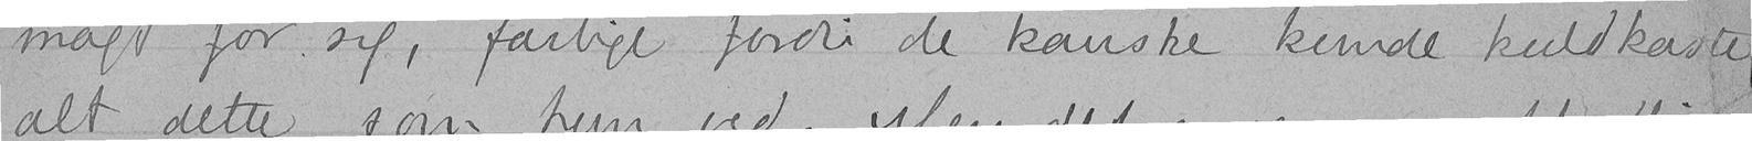magt for sig, farlige fordi de kanske kunde kuldkaste
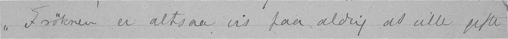"Frøknen er altsaa vis paa aldrig at ville gifte
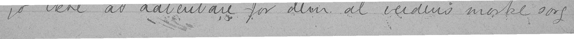jo ikke at aabenbare for dem al verdens mørke sorg
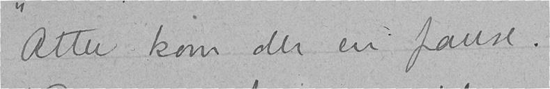Atter kom der en pause.
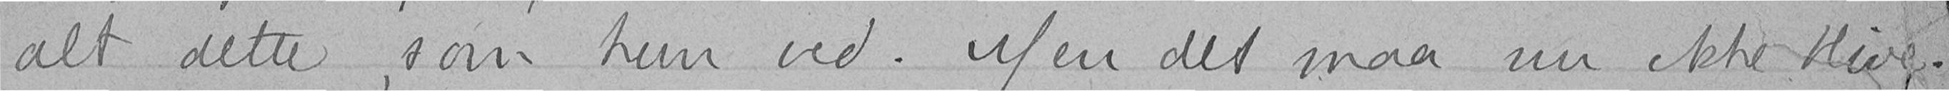alt dette, som hun ved. Men det maa nu ikke blive.
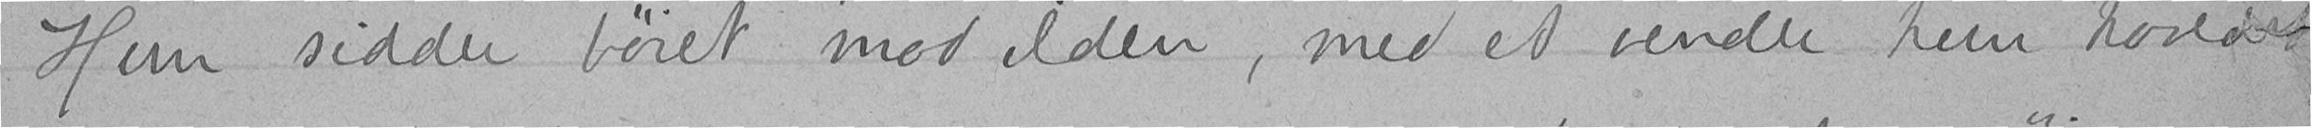Hun sidder bøiet mod ilden, med et vender hun hovedet
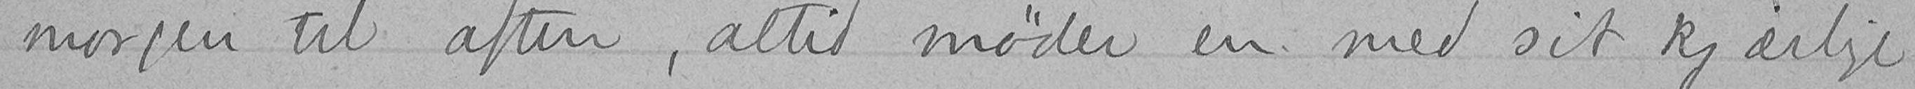morgen til aften, altid møder en med sit kjærlige
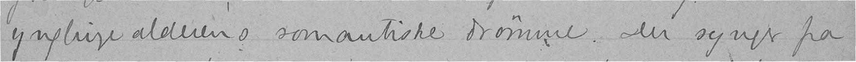ynglingealderens romantiske drømme. Der synger fra
